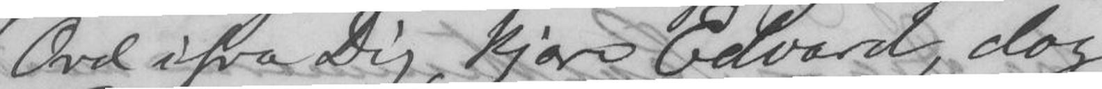Ord ifra Dig, kjære Edvard, dog
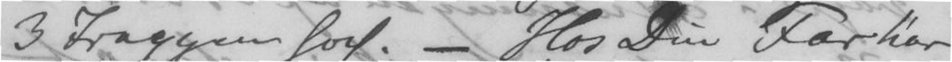3 Trappen fors. - Hos Din Far har
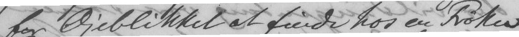for Øjeblikket at finde hos en Frøken
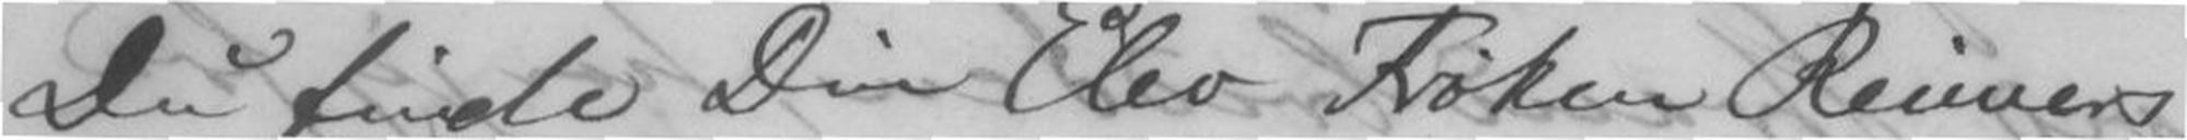Du finde Elev Frøken Reimers
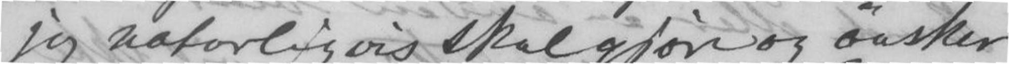jeg naturligvis skal gjøre og ønsker
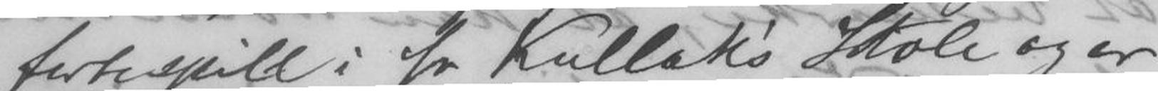fortespill i fr Kullak's Skole og er
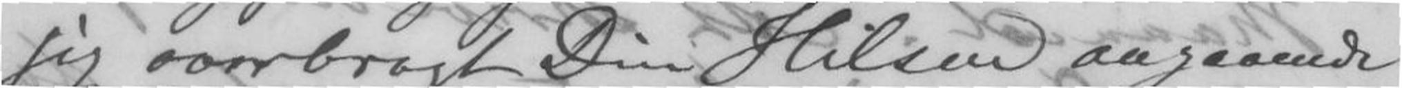jeg overbragt Din Hilsen angaaende
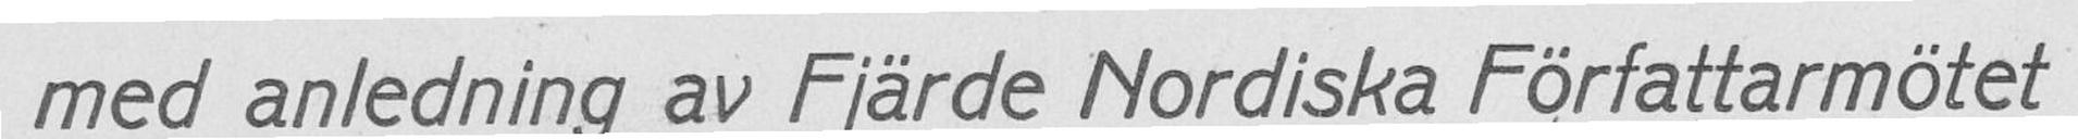med anledning av Fjärde Nordiska Författarmötet
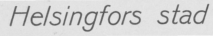Helsingfors stad
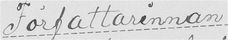Forfattarinnan
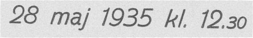28 maj 1935 kl. 12.30
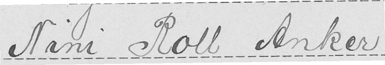Nini Roll Anker
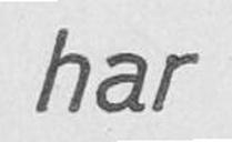har
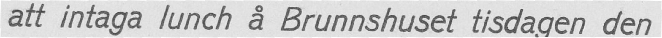att intaga lunch å Brunnshuset tisdagen den
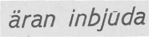ären inbjuda
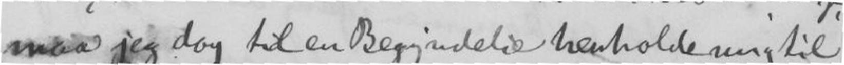maa jeg dog til en Begyndelse henholde mig til
